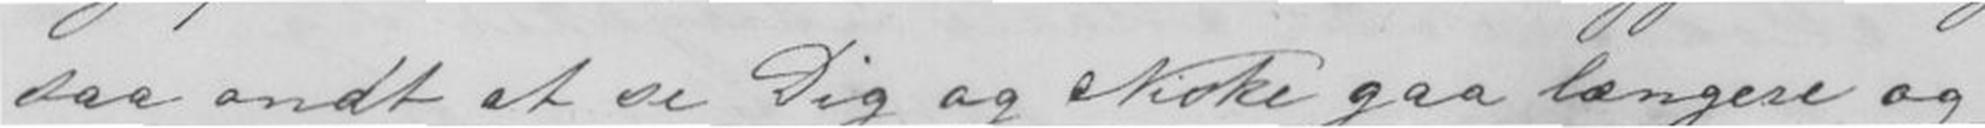saa ondt at se Dig og Niske gaa længere og
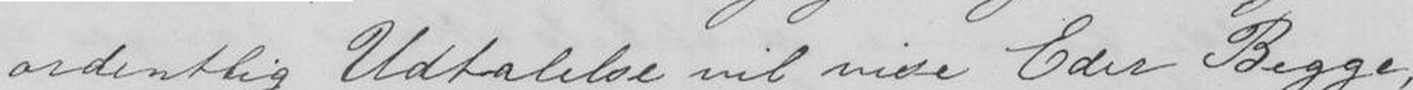ordentlig Udtalelse vil vise Eder Begge,
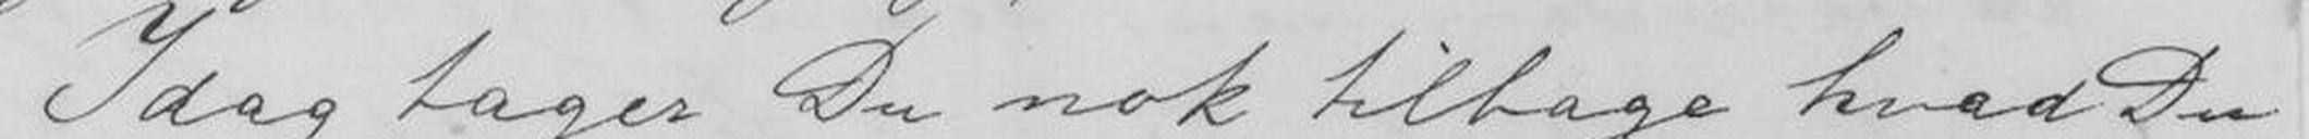I dag tager Du nok tilbage hvad Du
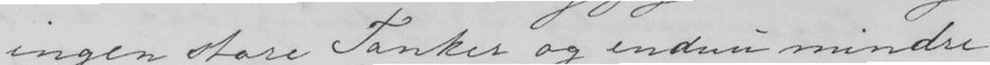ingen store Tanker og endnu mindre
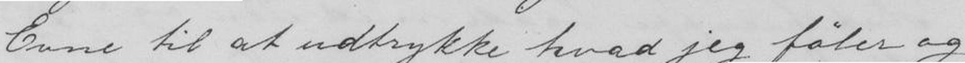Evne til at udtrykke hvad jeg føler og
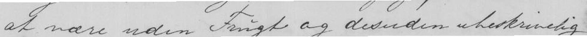at være uden Frugt og desuden ubeskrivelig
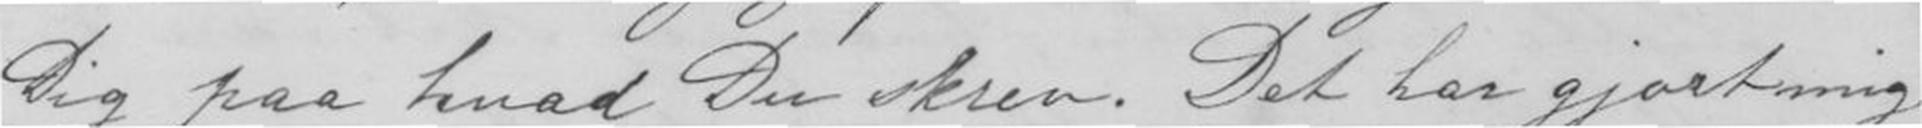Dig paa hvad Du skrev. Det har gjort mig
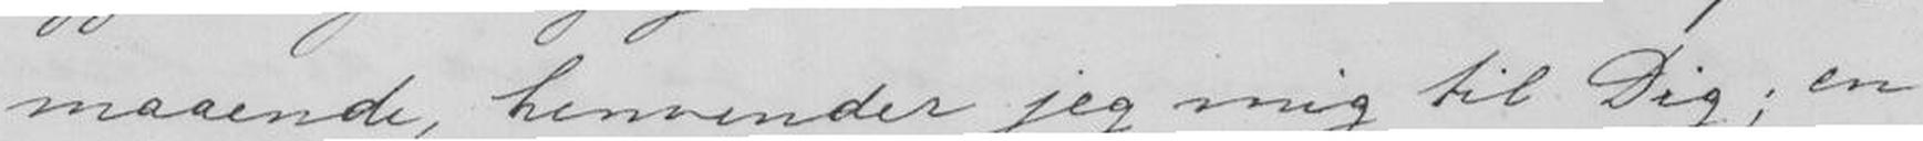maaende, henvender jeg mig til Dig; en
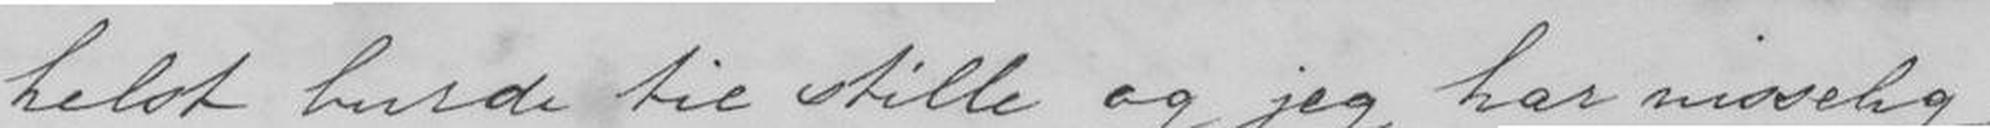helst burde tie stille og jeg har visselig
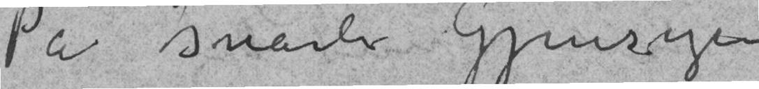På snarlig Gjensyn
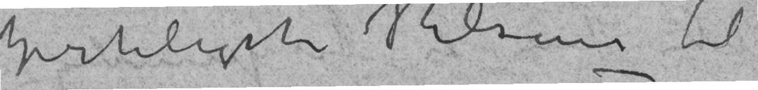hjerteligste Hilsener til
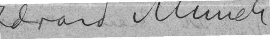Edvard Munch
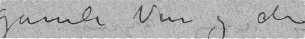gamle Ven og de
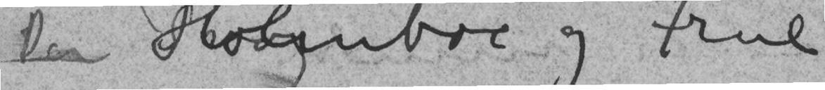Din Holmboe og Frue
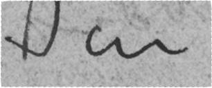Din
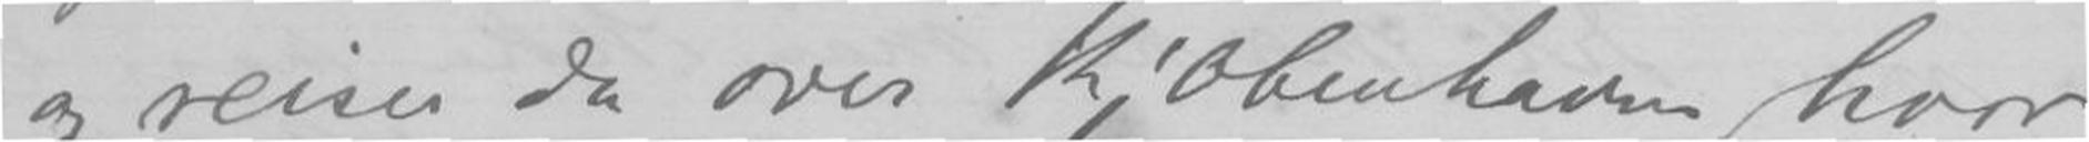og reiser da over Kjøbenhavn hvor
